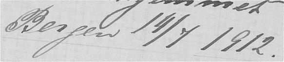Bergen 14/7 1912.
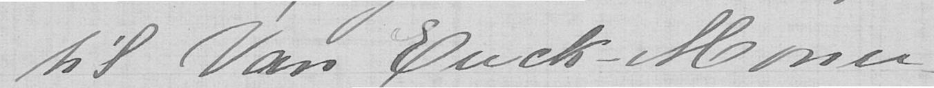til Van Euck-Monu-
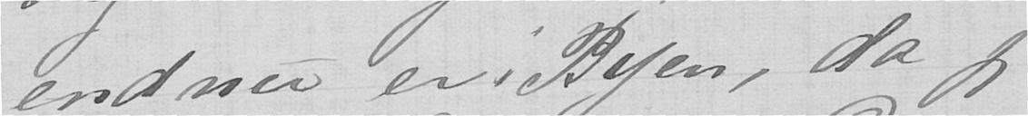endnu er Byen, da jeg
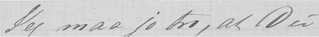Jeg maa jo tro, at Du
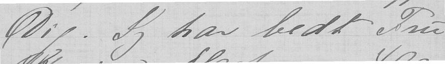Dig. Jeg har bedt Fru
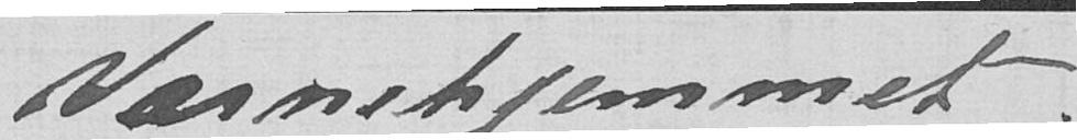Værnehjemmet
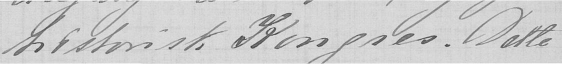historisk Kongres. Dette
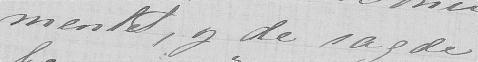mentet, og de sagde
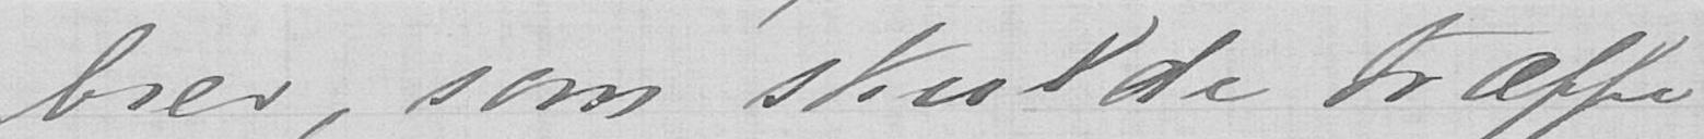brev, som skulde træffe
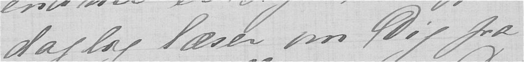daglig læser om Dig fra
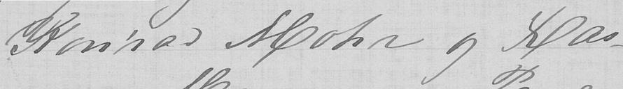Konrad Mohn og Ras-
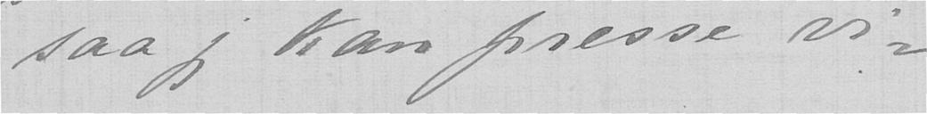saa jeg kan presse vi-
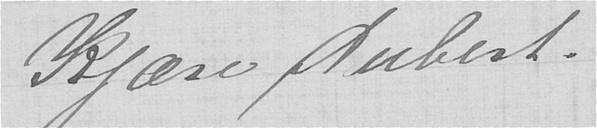Kjære Aubert.
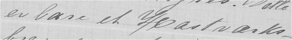er bare et Hastværks-
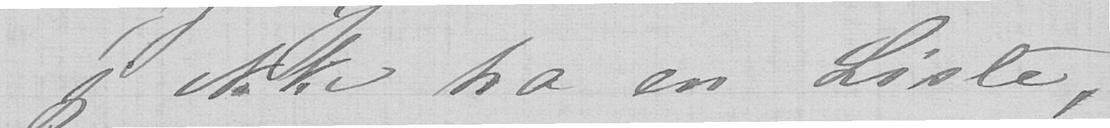jeg ikke ha en Liste,
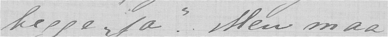begge "ja." Men maa
